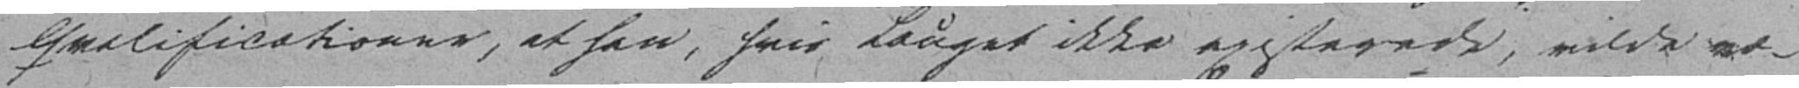Qvalificationer, at han, hvis Lauget ikke existerede, vilde væ-
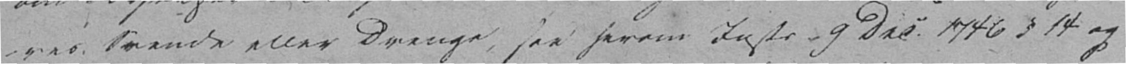res Svende eller Drenge, see herom Instr. 9 Dec. 1746 § 14 og
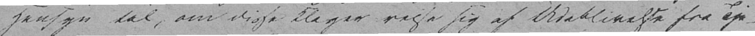Hensyn til, om disse Klager reise sig af Udeblivelse fra Tje-
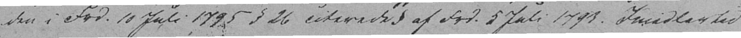den i Frd. 10 Juli 1795 § 26 citerede § af Frd. 5 Juli 1793. Imidlertid
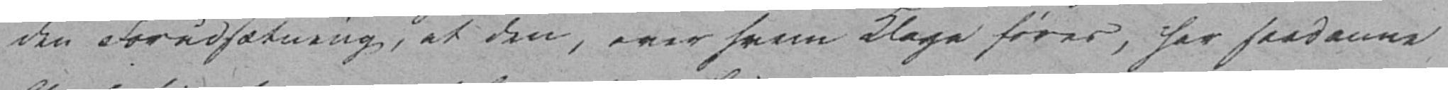den Forudsætning, at den, over hvem Klage føres, har saadanne
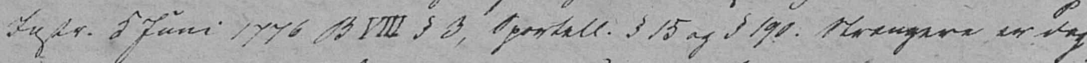Instr. 5 Juni 1776 B VIII § 3, Sportell. § 15 og § 190. Strengere er dog
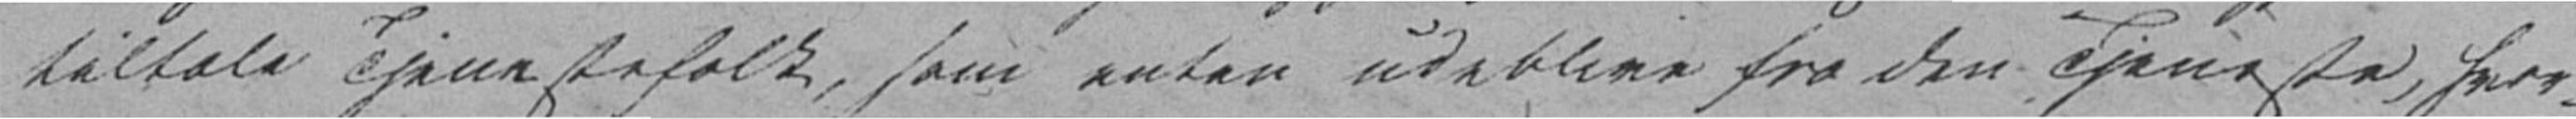tiltale Tjenestefolk, som enten udeblive fra den Tjeneste, hvor-
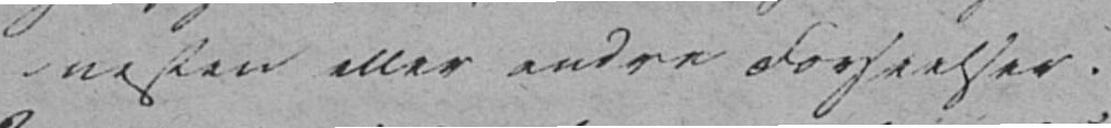nesten eller andre Forseelser.
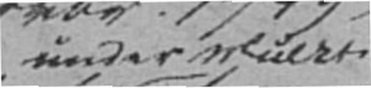under Mulkt
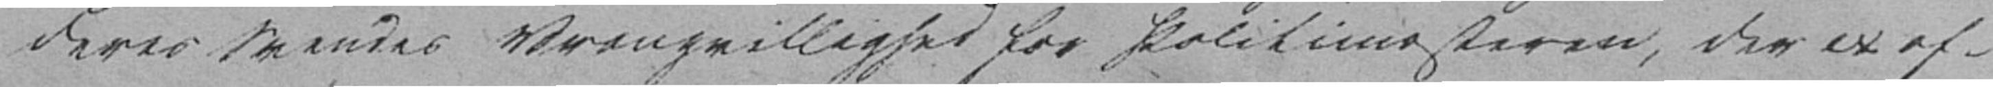deres Svendes Vrangvillighed for Politimesteren, der ex of-
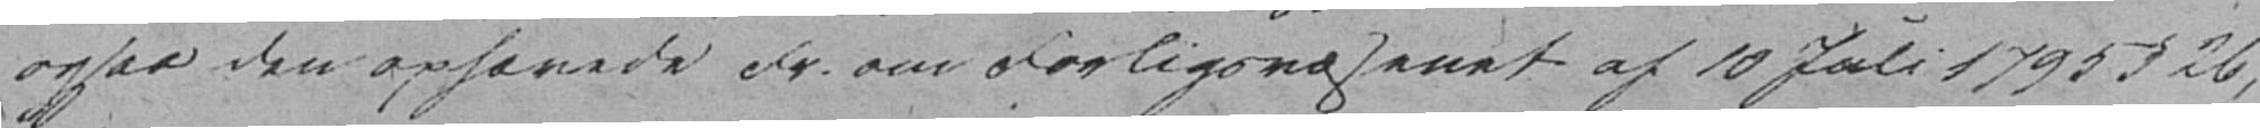ogsaa den ophævede Fr. om Forligsvæsenet af 10 Juli 1795 § 26,
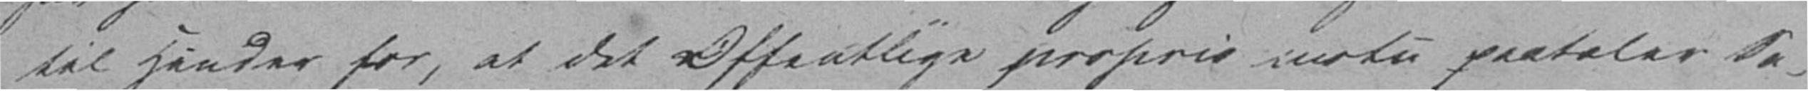til Hinder for, at det Offentlige proprio motu paataler Sa-
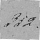352.
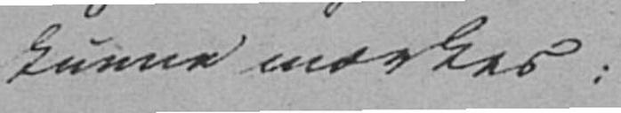kunne mærkes :
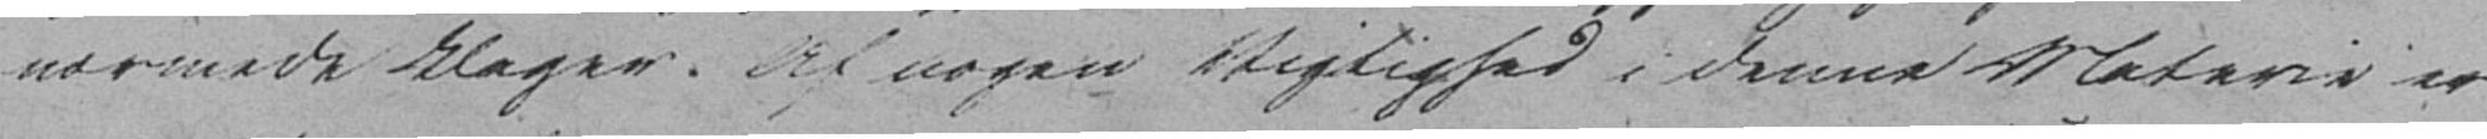nærmede klager. Af nogen Vigtighed i denne Materie er
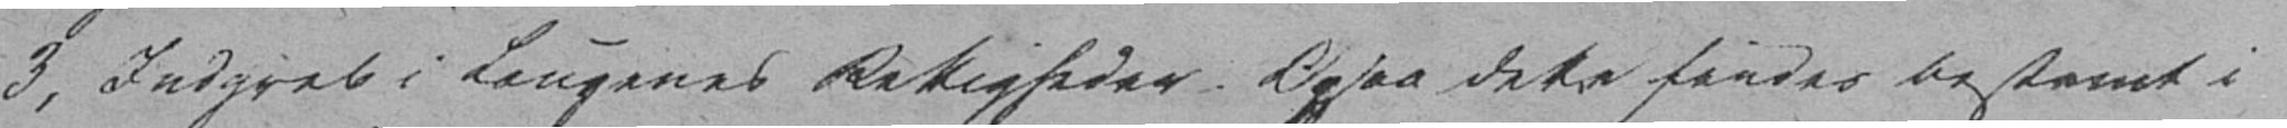3, Indgreb i Laugenes Rettigheder. Ogsaa dette findes bestemt i
